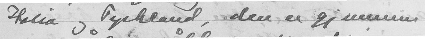Italia og Tyskland, den er gjennom
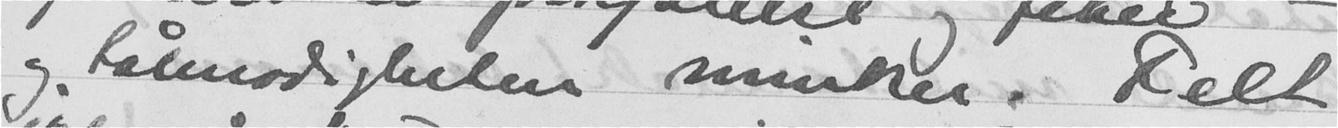og tålmodigheten minker. Felt
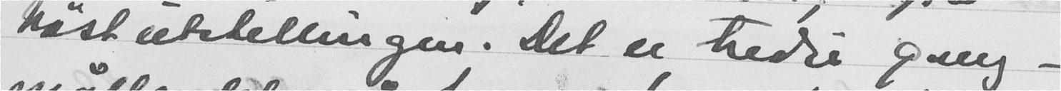høstutstillingen. Det er tredje gang -
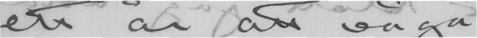ett år att våga
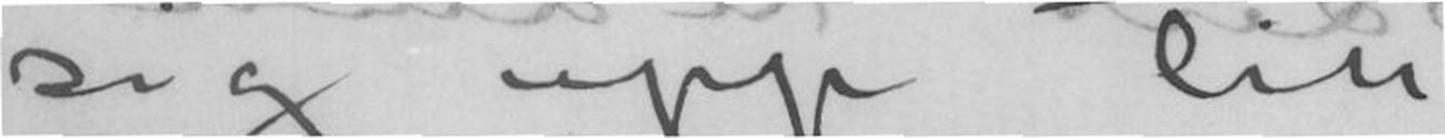sig upp till
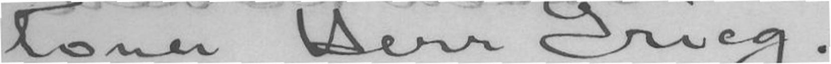toner Herr Grieg.
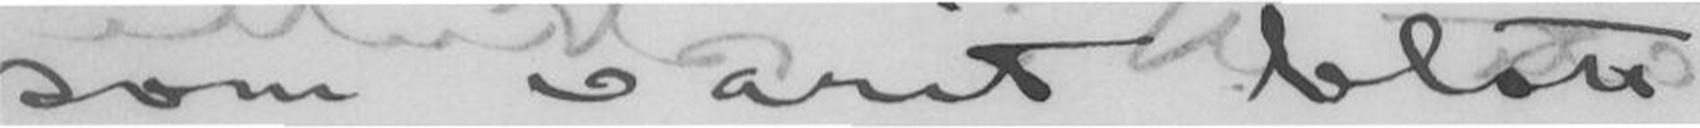som varit blott
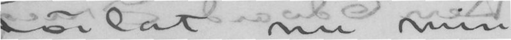Forlåt nu min
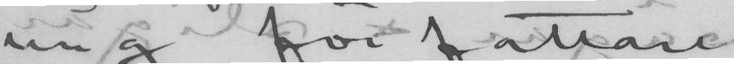ung forfattare
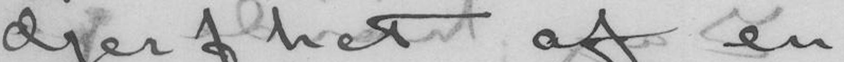djerfhet af en
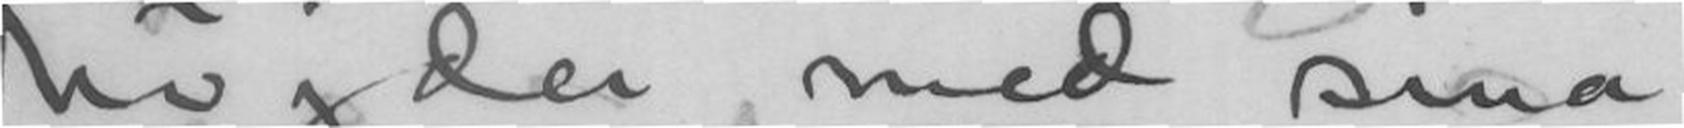højder med sina
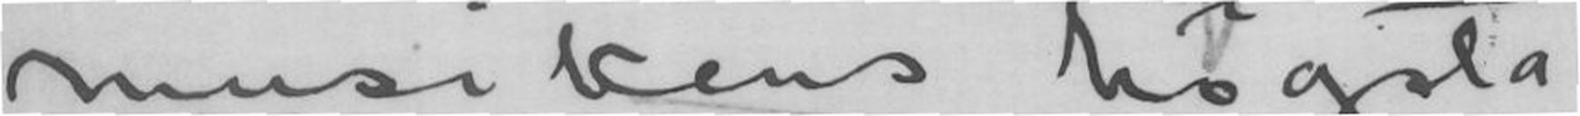musikens høgsta
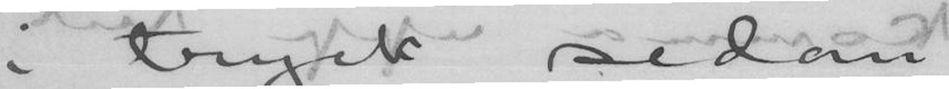i "tryck" sedan
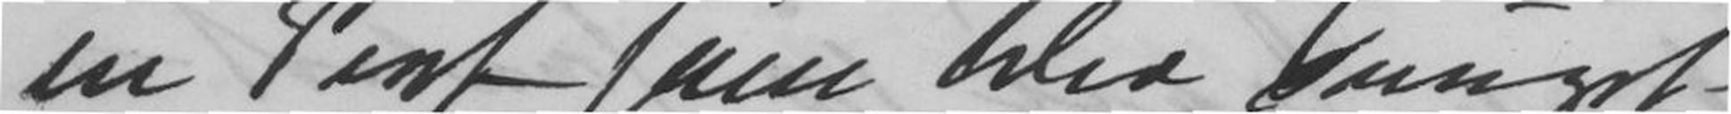en text som blev sunget
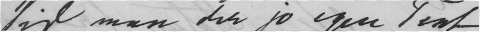tid maa der jo igen Text
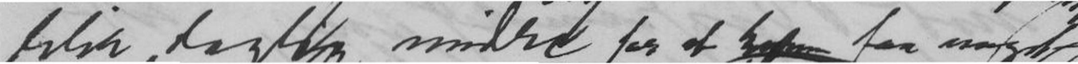blir daglig mindre for at kunne faa noget
